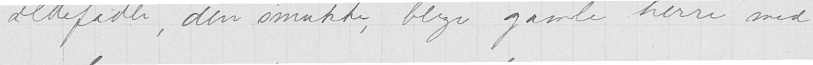oldefader, den smukke, blyge gamle herre med
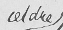ældre
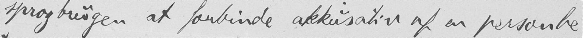sprogbrugen at forbinde akkusativ af en personbe-
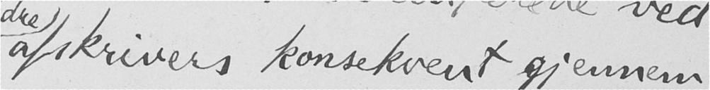afskrivers konsekvent gjennem-
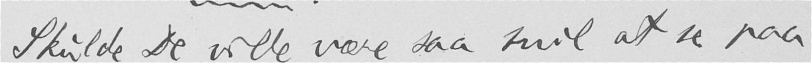Skulde De ville være saa snil at se paa
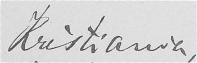Kristiania,
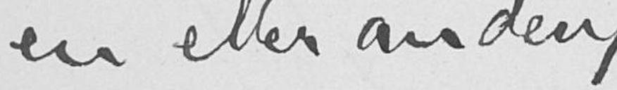en eller anden
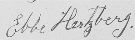Ebbe Hertzberg.
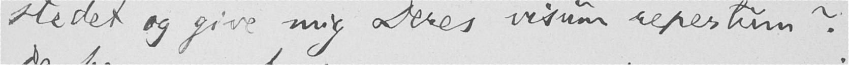stedet og give mig Deres visum repertum?
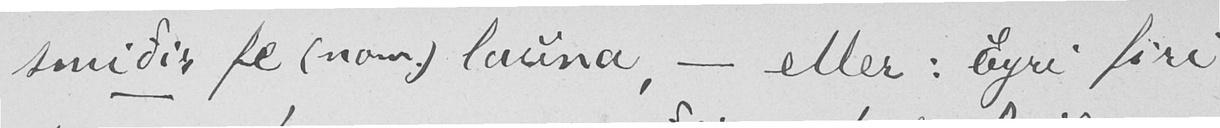smiðir fe (nom.) launa, - eller: Eyri firi
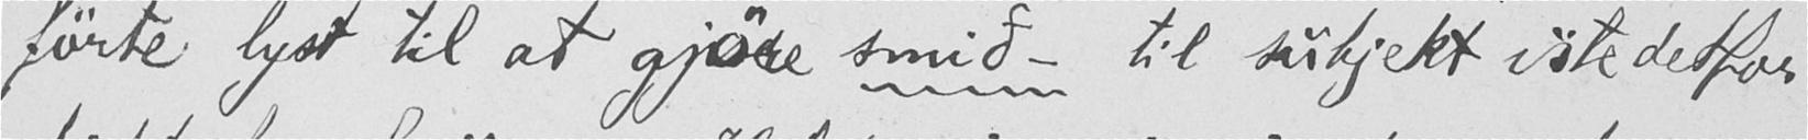førte lyst til at gjøre smið- til subjekt istedetfor
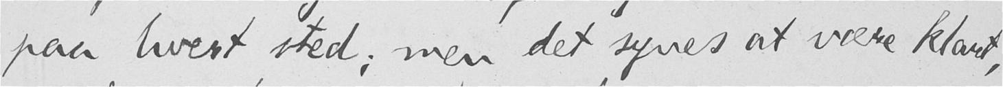paa hvert sted; men det synes at være klart,
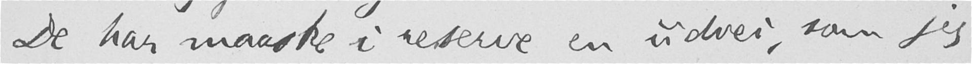De har maaske i reserve en udvei, som jeg
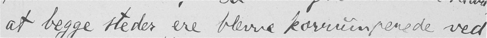at begge steder ere blevne korrumperede ved
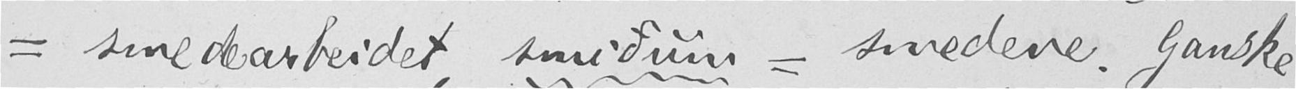= smedearbeidet, smiðum = smedene. Ganske
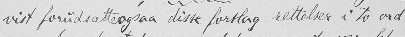vist forudsætter ogsaa disse forslag rettelser i to ord
